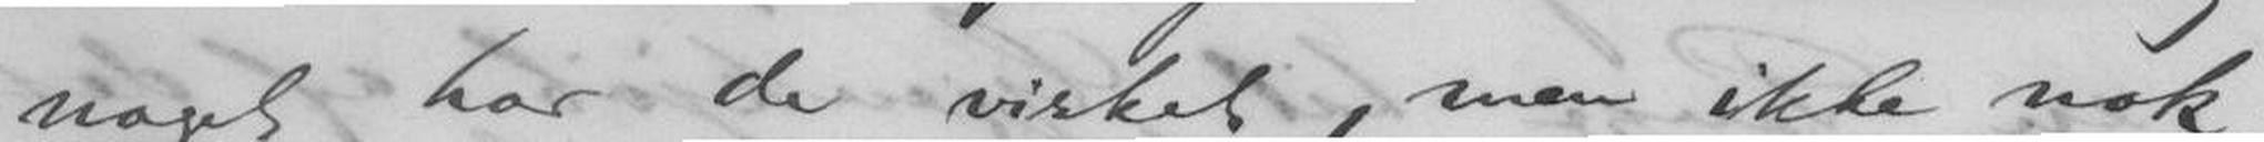noget har de virket, men ikke nok.
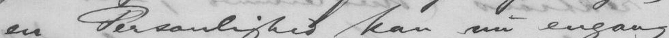en Personlighed kan nu engang
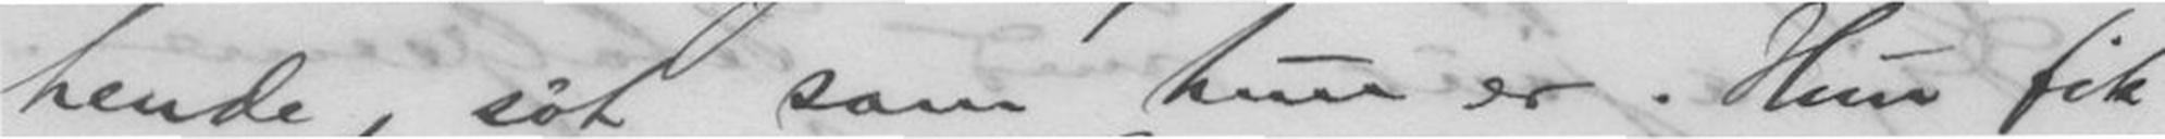hende, søt som hun er. Hun fik
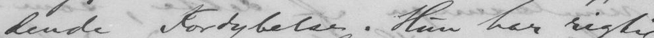dende Fordypelse. Hun har rigtig
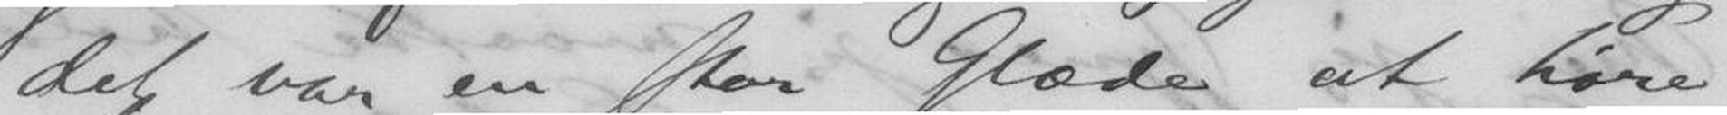det var en stor Glæde at høre
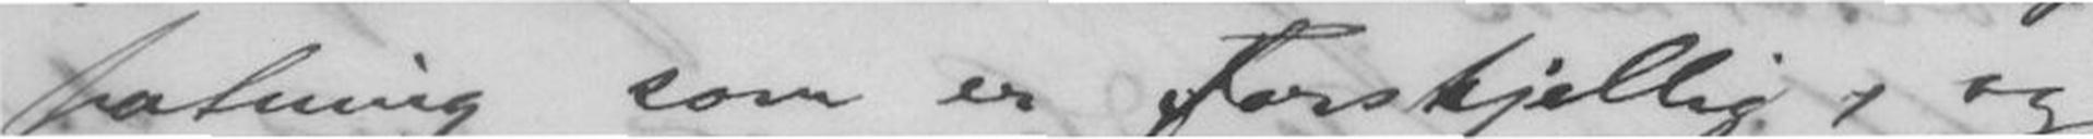fatning som er forskjellig, og
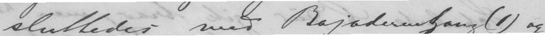sluttedes med Bajaderentgang (1) og
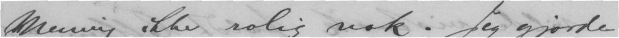Mening ikke rolig nok. Jeg gjorde
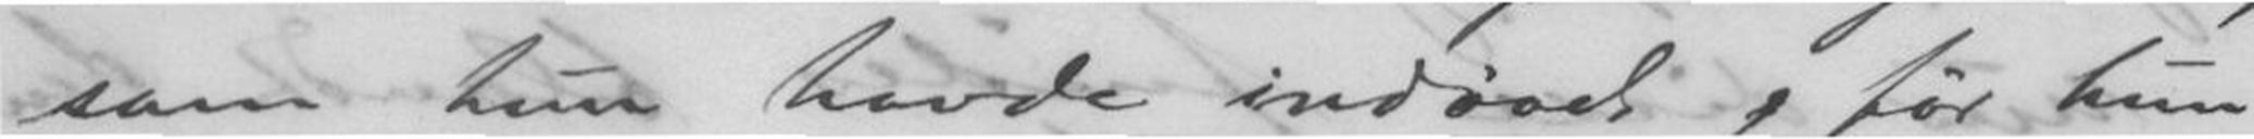som hun havde indøvet, før hun
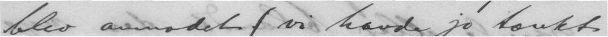blev anmodet (vi havde jo tænkt
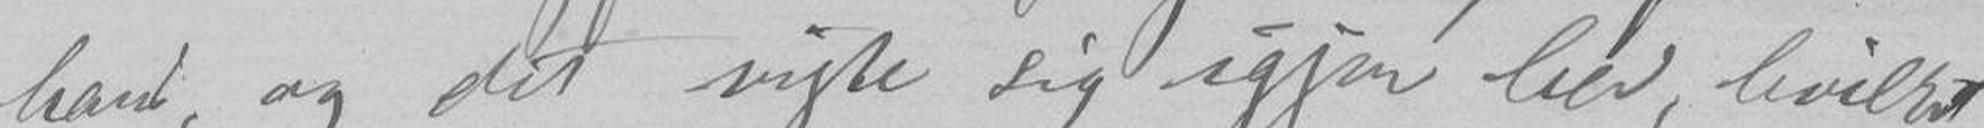hans, og det reiste sig igjen her, hvilket
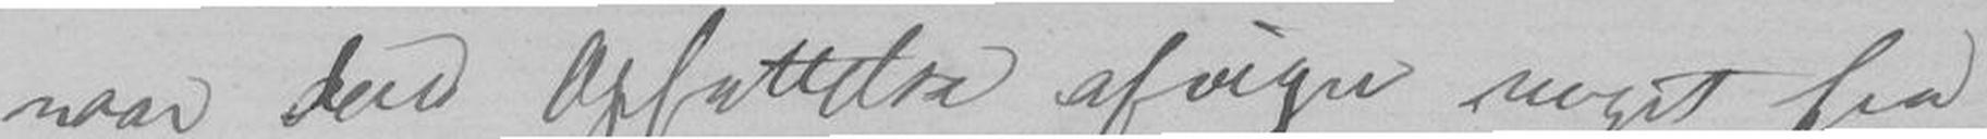naar dens Opfattelse afviger noget fra
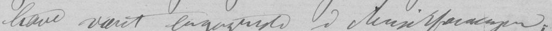have været engaserede i Musikforeningen;
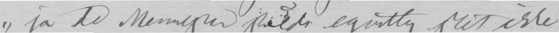ja de Mennesker skulde egentlig slet ikke
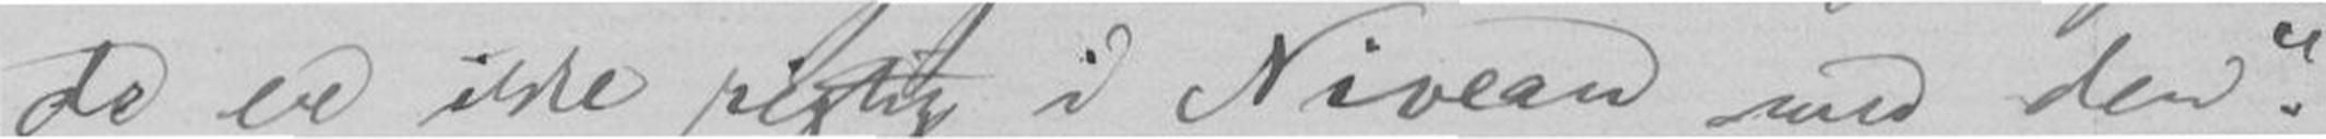de ere ikke rigtig i Niveau med den.
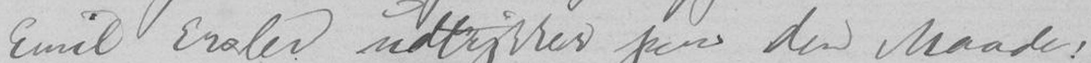Emil Erslev udtrykker paa den Maade;
